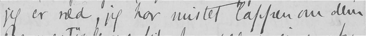jeg er ræd, jeg har mistet lappen om dem
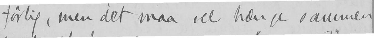førlig, men det maa vel hænge sammen
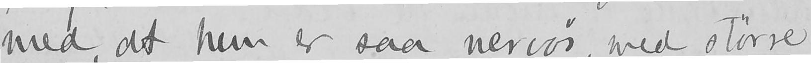med, at hun er saa nervøs, med større
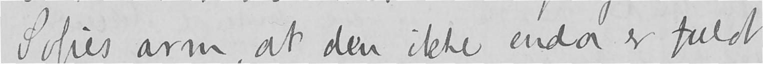Sofies arm, at den ikke enda er fuldt
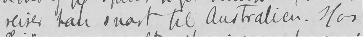reiser han snart til Australien. Hos
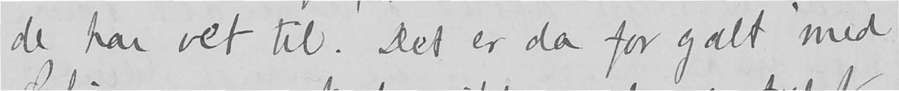de har ret til. Det er da for galt med
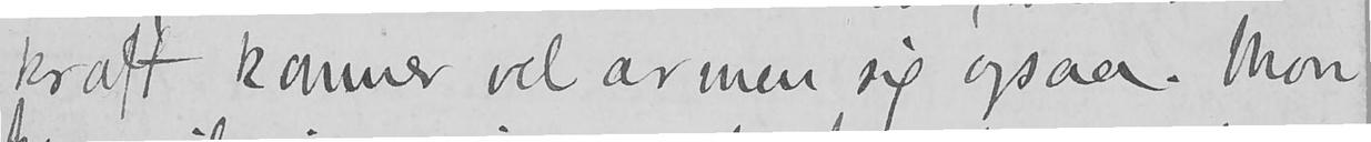kraft kommer vel armen sig ogsaa. Mon
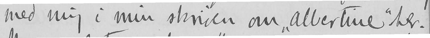med mig i min skriven om "Albertine" her.
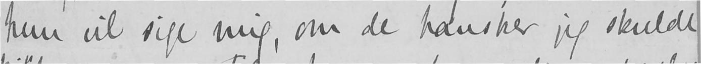hun vil sige mig, om de hansker jeg skulde
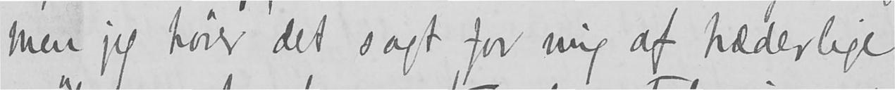men jeg hører det sagt for mig af hæderlige
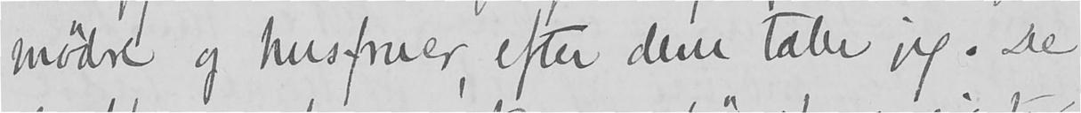mødre og husfruer, efter dem taler jeg. De
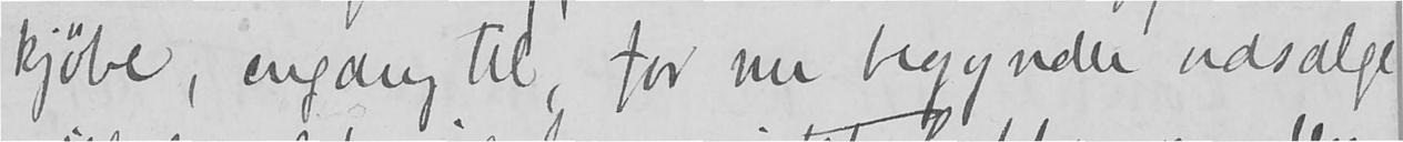kjøbe, engang til, for nu begynder udsalge
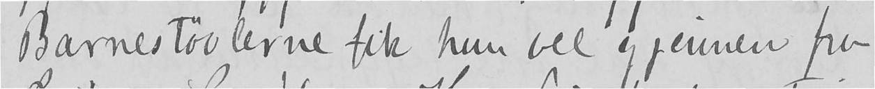Barnestøvlerne fik hun vel og reimen fra
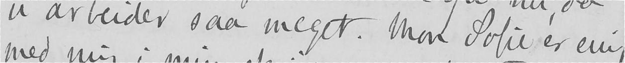vi arbeider saa meget. Mon Sofie er enig
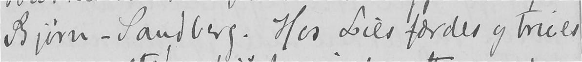Bjørn-Sandberg. Hos Lies færdes og trues
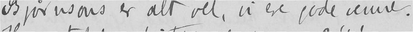Bjørnsons er alt vel, vi ere gode venne.
